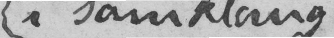i samklang
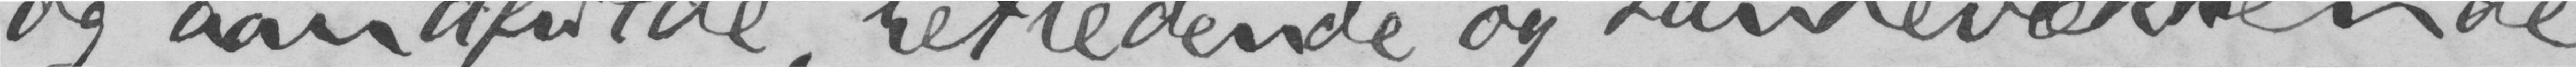og aandfulde, retledende og tankevækkende
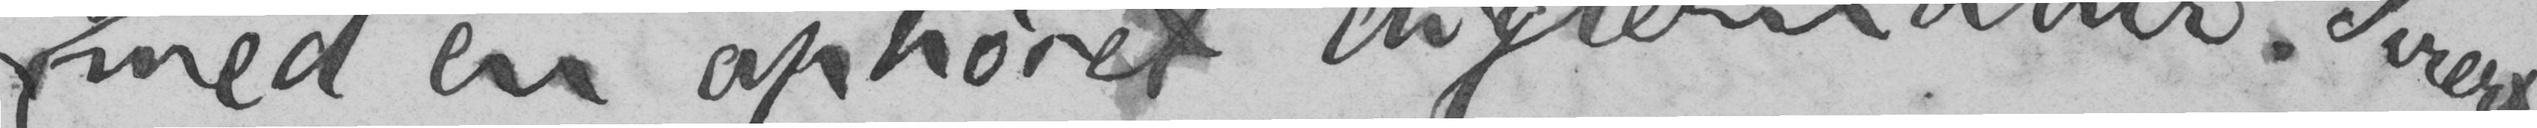med en ophøiet digternatur. Tvert-
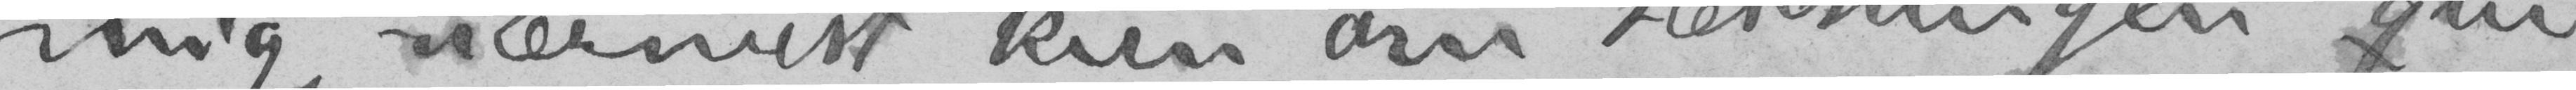mig, nærmest kun om sætningen «qui
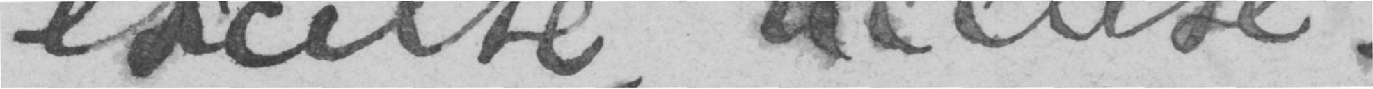excuse, aceuse».
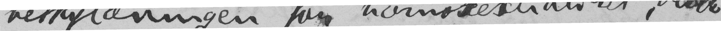beskyldningen for homosexualitet, veier
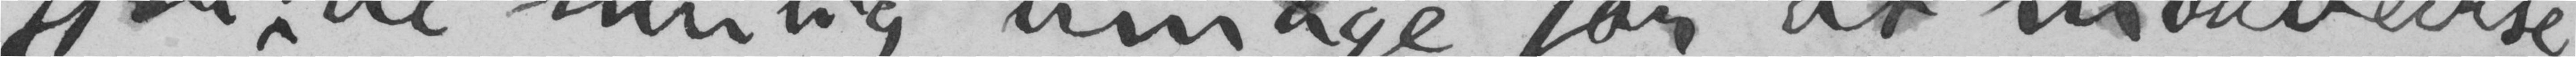gjør sig al mulig umage for at modbevise
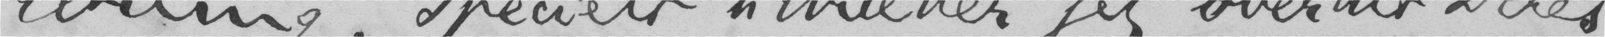retning. Specielt tiltræder jeg overalt Deres
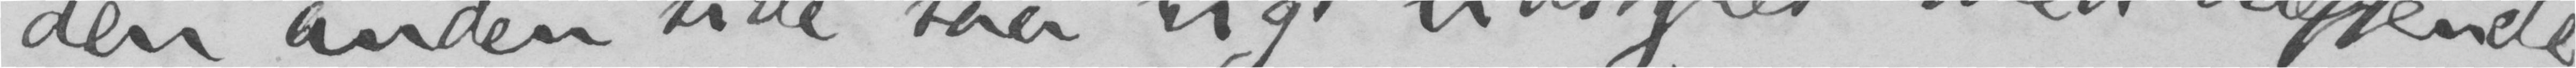den anden side saa rigt udstyret med træffende
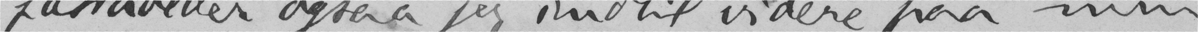fastholder ogsaa jeg indtil videre paa min
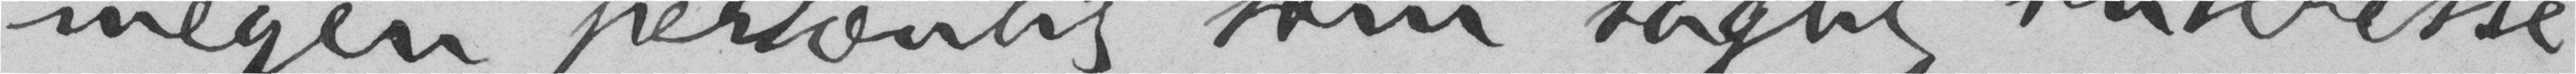megen personlig som saglig interesse.
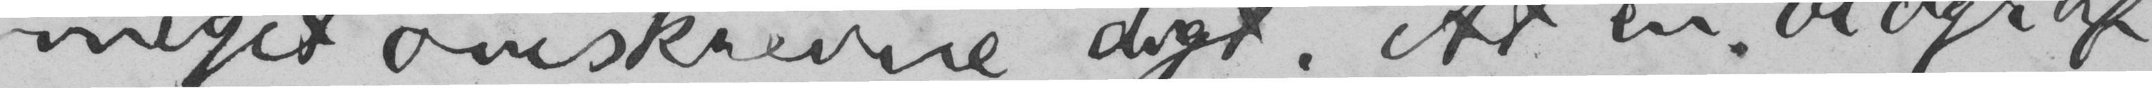meget omskrevne digt. At en biograf
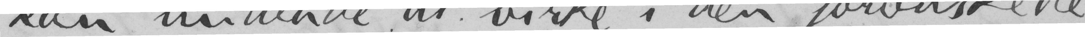kan undlade at virke i den forønskede
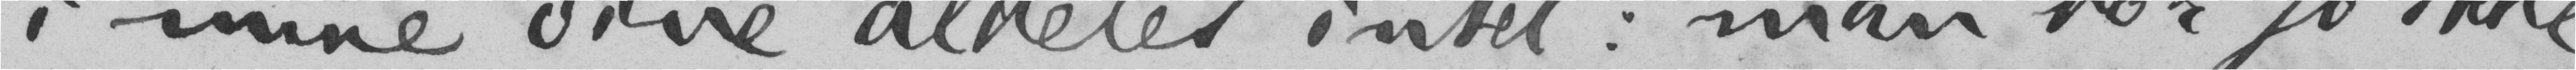i mine øine aldeles intet: man bør jo ikke
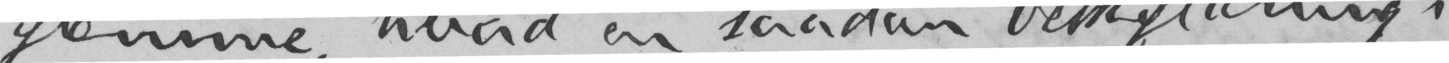glemme, hvad en saadan beskyldning i
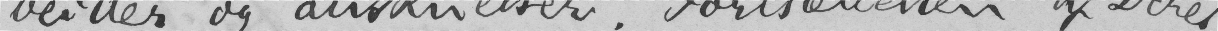beider og anskuelser. Fortsættelsen af Deres
